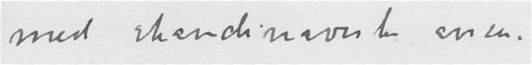med skandinaviske aviser.
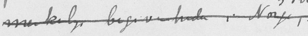merkelige begivenheder i Norge,
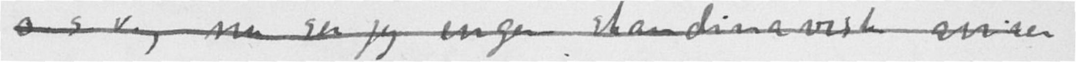o.s.v., nu ser jeg ingen skandinaviske aviser
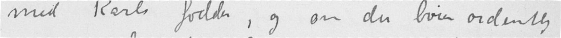med Karls fødder, og om du bøier ordentlig
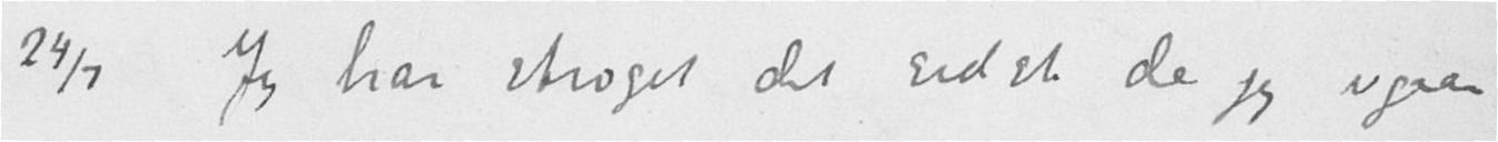24/7 Jeg har strøget det sidste da jeg i gaar
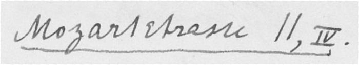Mozartstrasse II, IV.
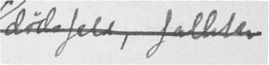dødsfald, falliter
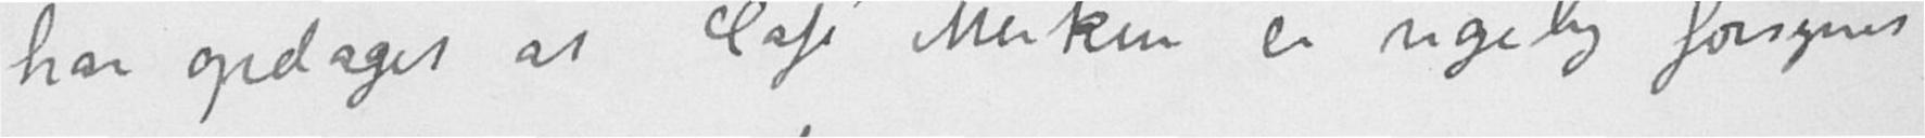har opdaget at Café Merkur er rigelig forsynet
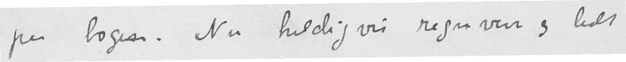paa bogen. Nu heldigvis regnveir og lidt
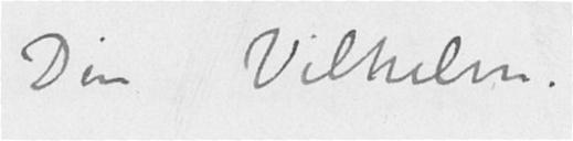Din Vilhelm.
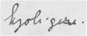kjøligere.
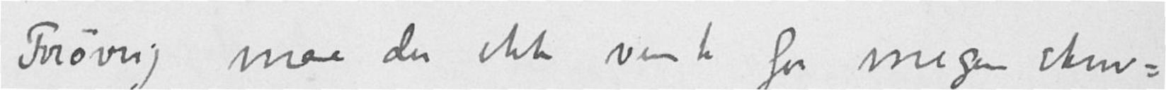Forøvrig maa du ikke vente for megen skriv-
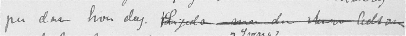paa dem hver dag. Ligedan maa du skrive lidt om
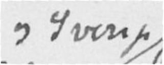og Sverige
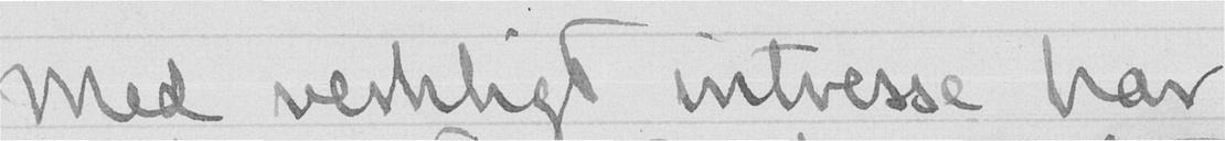Med verkligt interesse har
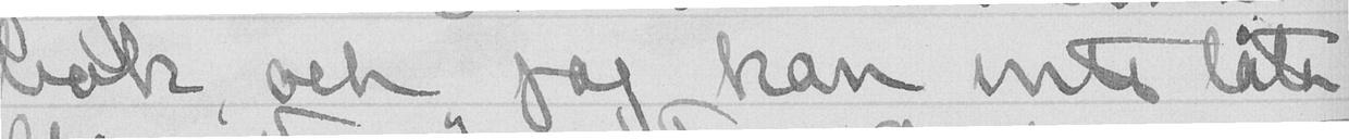bok, och jag kan inte låte
